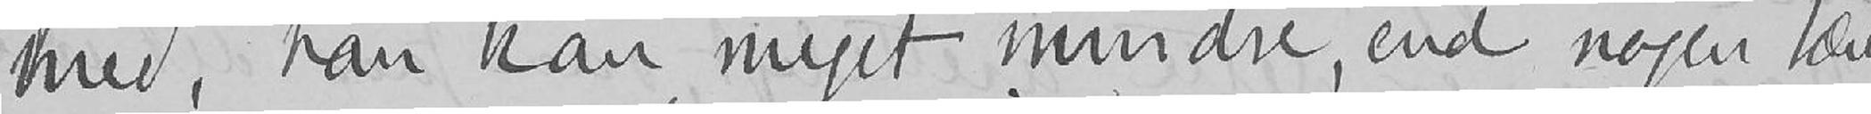med, han kan meget mindre, end nogen tænk
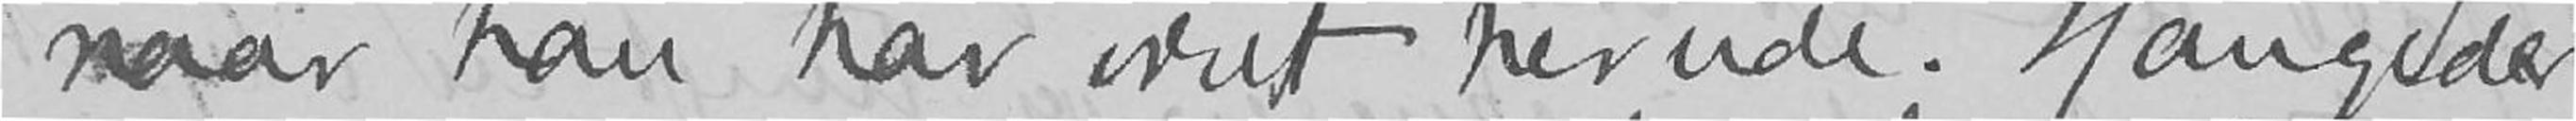naar han har været herude. Han gider
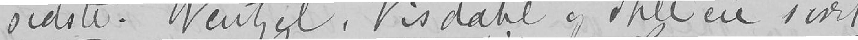sidste. Wentzel, Visdahl og Ihle ere svært
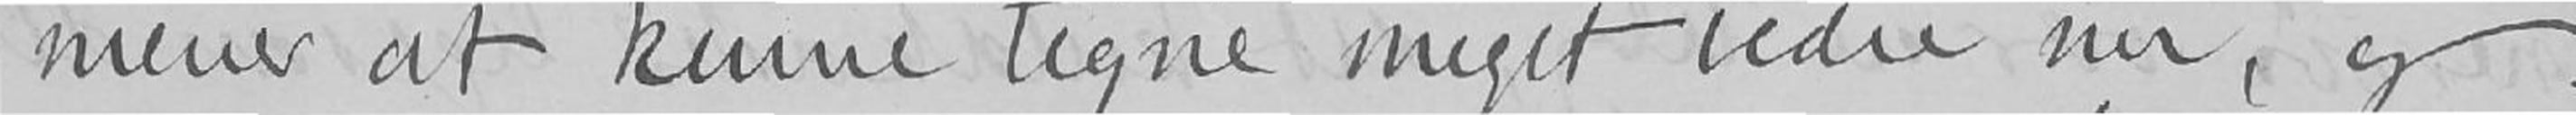mener at kunne tegne meget bedre nu, og
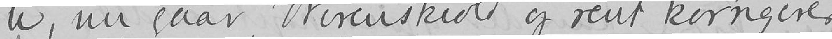te, nu gaar Werenskiold og rent korrigerer
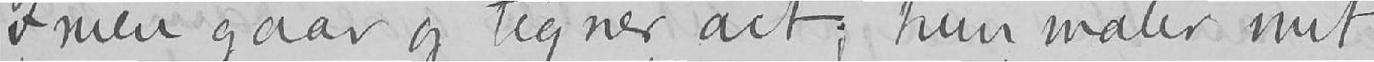Fruen gaar og tegner act; hun maler mit
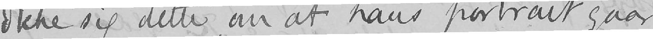Ikke sig dette om at hans portrait gaar
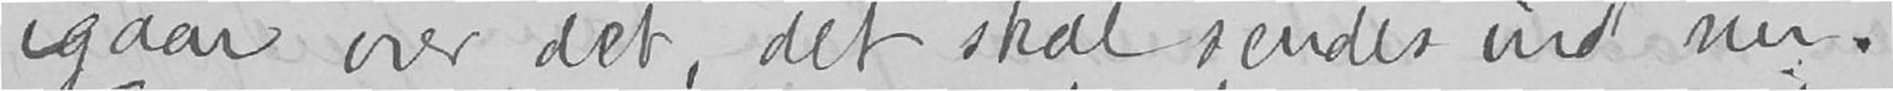igaar over det, det skal sendes ind nu.
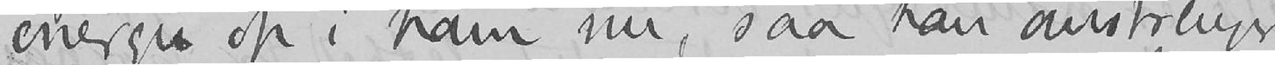energi op i ham nu, saa han anstrenger
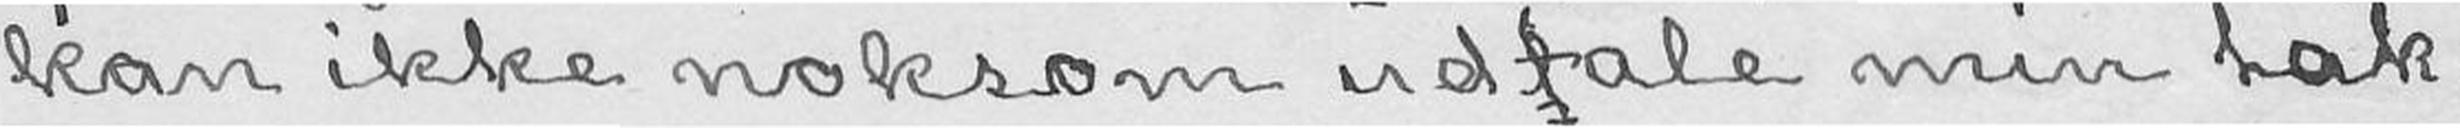kan ikke noksom udtale min tak-
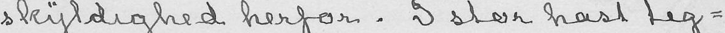skyldighed herfor. I stor hast teg-
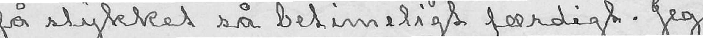få stykket så betimeligt færdigt. Jeg
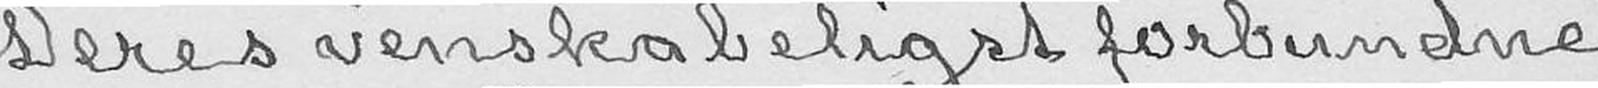Deres venskabeligst forbundne
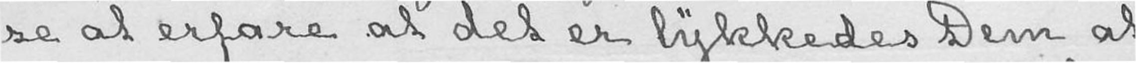se at erfare at det er lykkedes Dem at
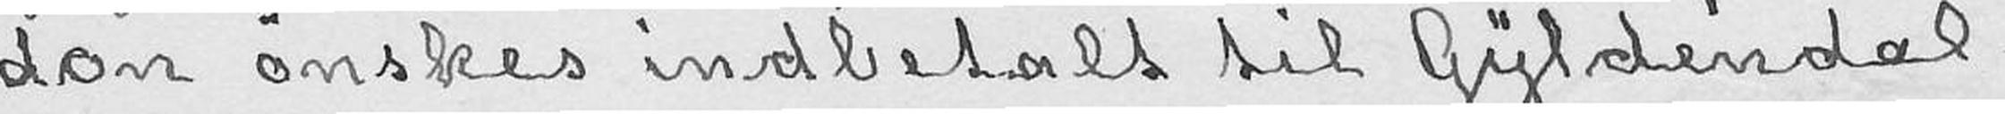don ønskes indbetalt til Gyldendal-
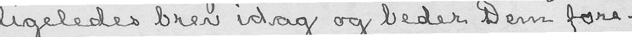ligeledes brev idag og beder Dem fore-
